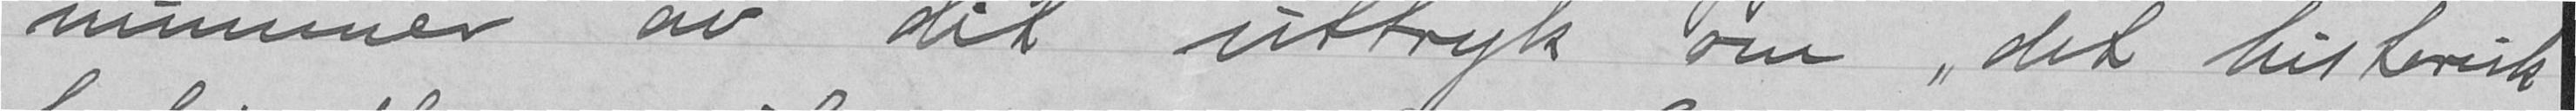nummer av dit uttryk om det historisk
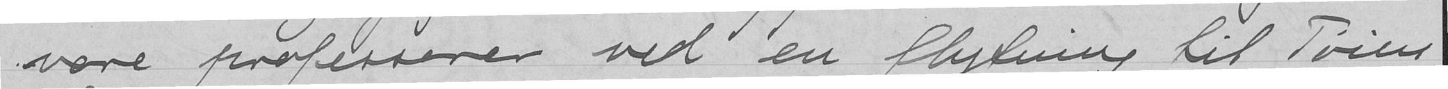vore professorer ved en flytning til Tøien
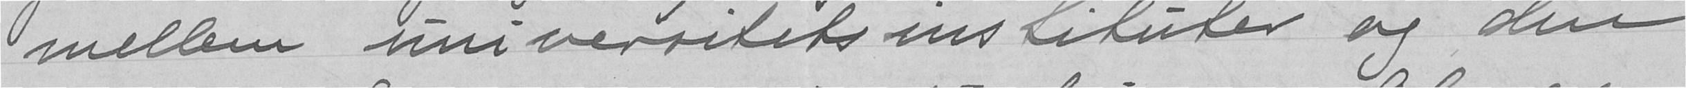mellem universitets instituter og den
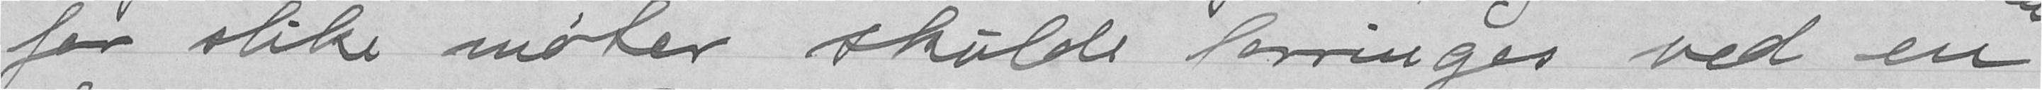for slike møter skulde forringes ved en
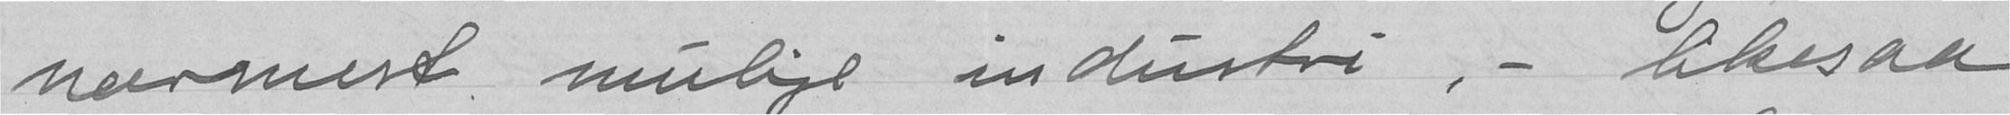nærmest mulige industri, - likesaa
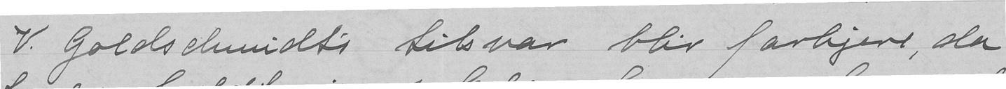V. Goldschmidts tilsvar blev forkjere, da
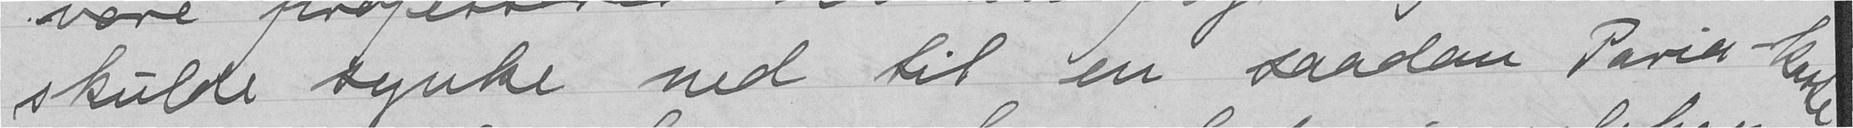skulde synke ned til en saadan Paria-kaste
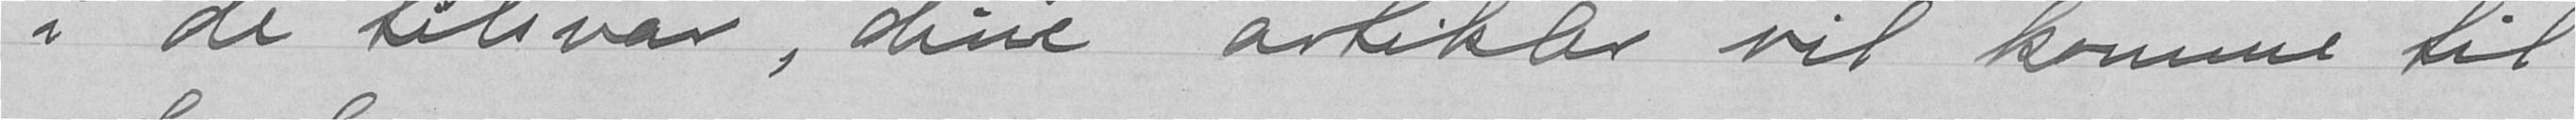i de tilsvar, dine artikler vil komme til
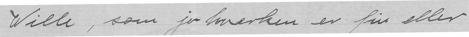Wille, som jo hverken er fin eller
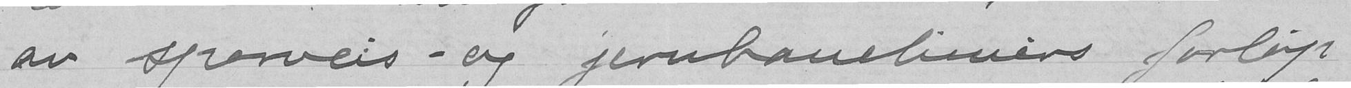av sporveis- og jernbaneliners forløp
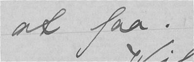at faa.
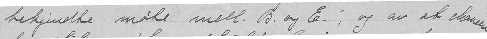bekjendte møte mell. B. og E., og av at chance
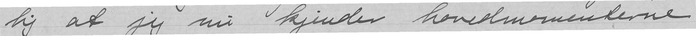lig at jeg nu kjender hovedmomenterne
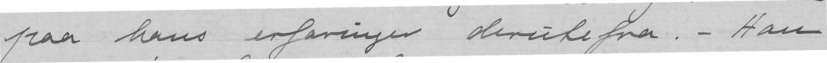paa hans erfaringer derute fra. - Han
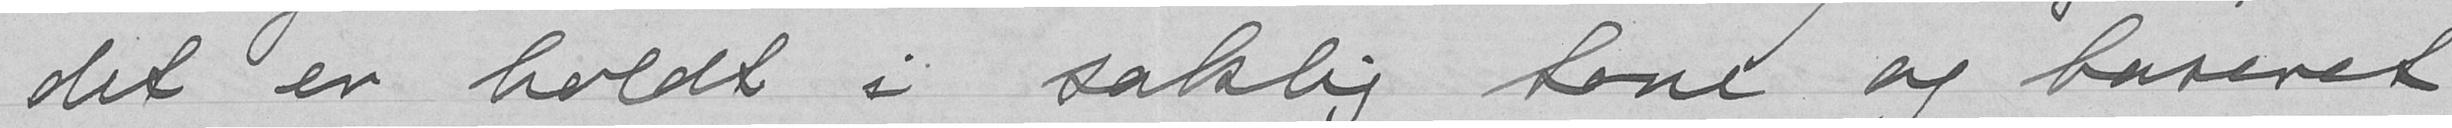det er holdt i saklig tone og baseret
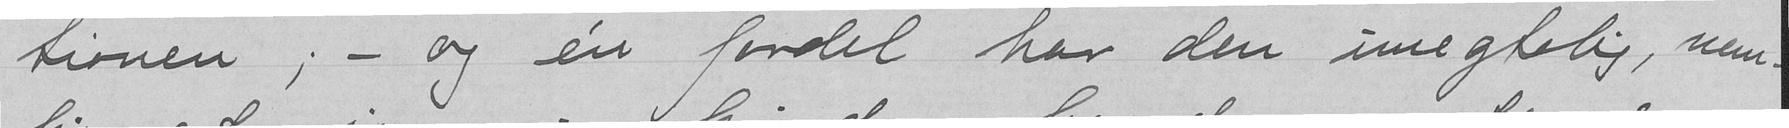tionen, - og en fordel har den unegtelig, nem-
